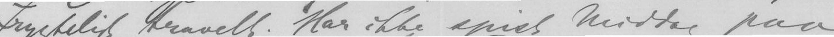Frygtelig travelt. Har ikke spist Middag paa
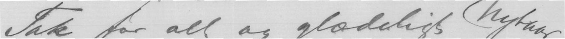Tak for alt og glædeligt Nytaar!
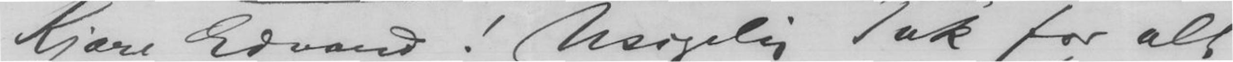Kjære Edvard! Usigelig Tak for alt
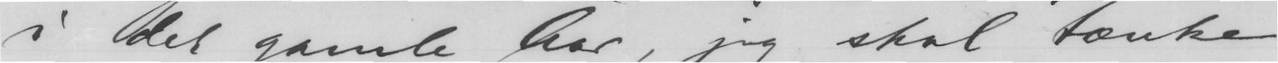i det gamle Aar, jeg skal tænke
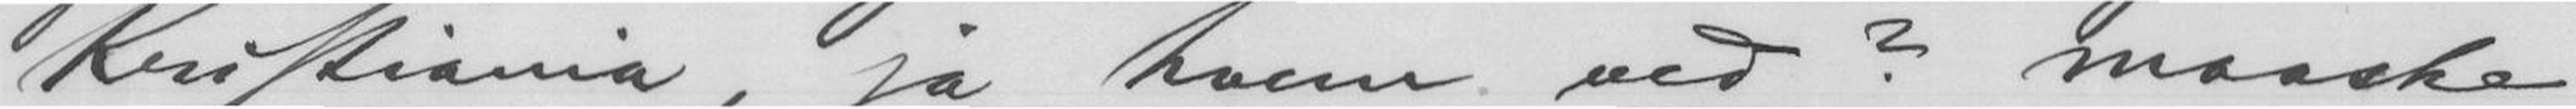Kristiania, ja hvem ved? maaske
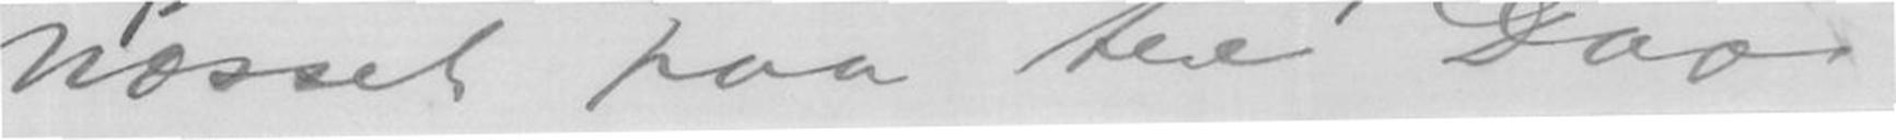Næsset paa tre Dage.
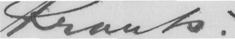Frants.
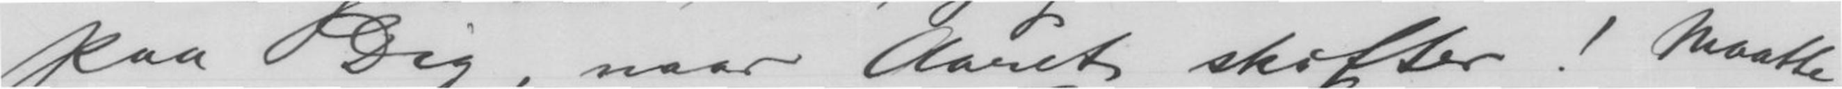paa Dig, naar Aaret skifter! Maatte
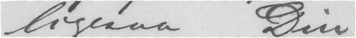ligesaa Din
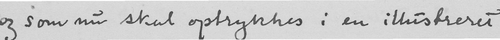og som nu skal optrykkes i en illustreret
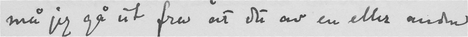må jeg gå ut fra at du av en eller anden
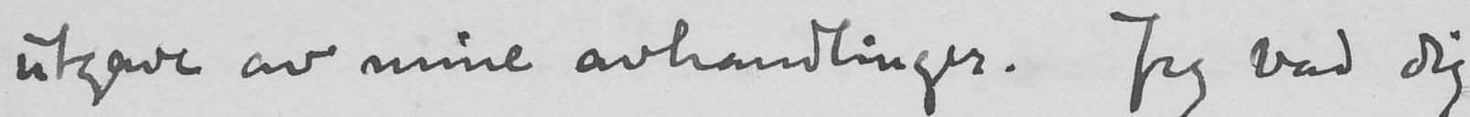utgave av mine avhandlinger. Jeg bad dig
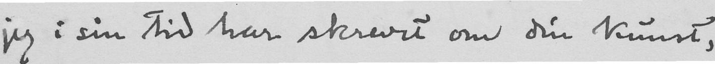jeg i sin tid har skrevet om din kunst,
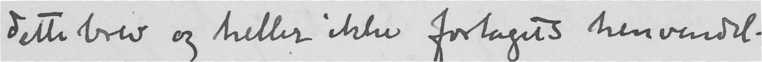dette brev og heller ikke forlagets henvendel-
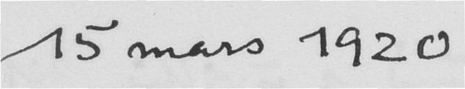15 mars 1920
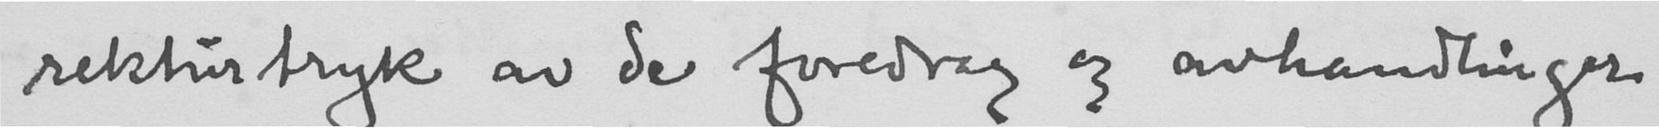rekturtryk av de foredrag og avhandlinger
